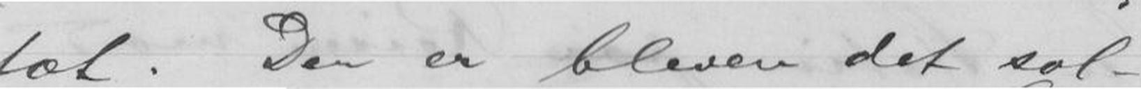tæt. Der er bleven det sol-
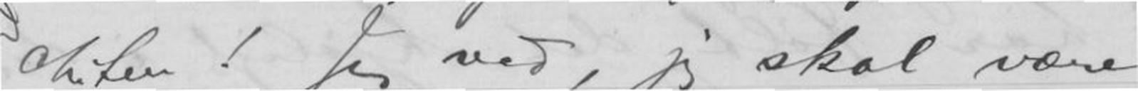chiten! Jeg ved, jeg skal være
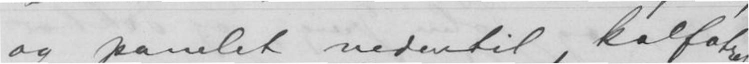og panelet nedentil kalfatret
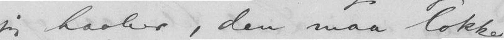jeg haaber, den maa lokke
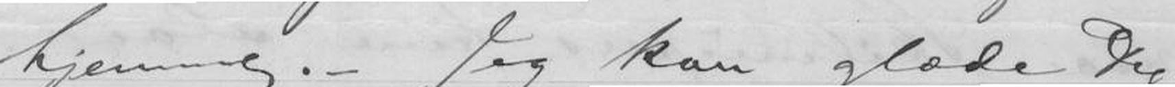hjemme. - Jeg kan glæde Dig
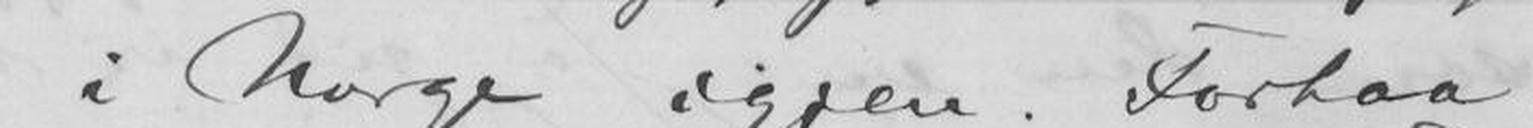me i Norge igjen. Forhaa-
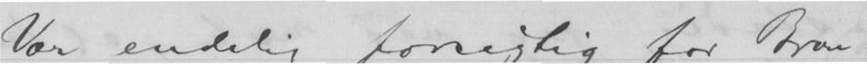Vær endelig forsigtig for Bron-
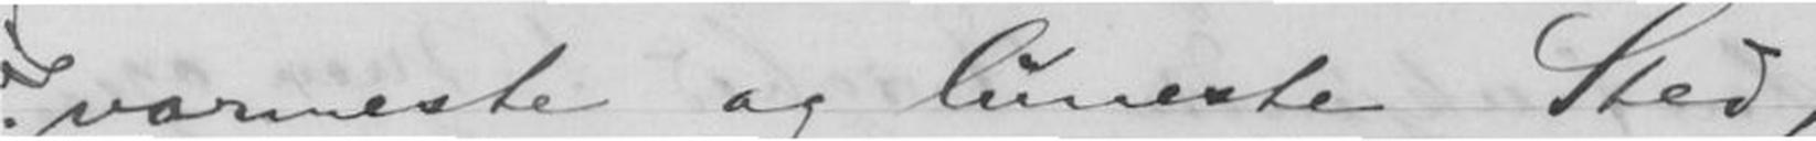varmeste og luneste Sted,
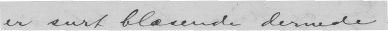er surt blæsende dernede.
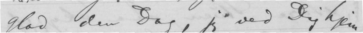glad den Dag, jeg ved Dig hjem-
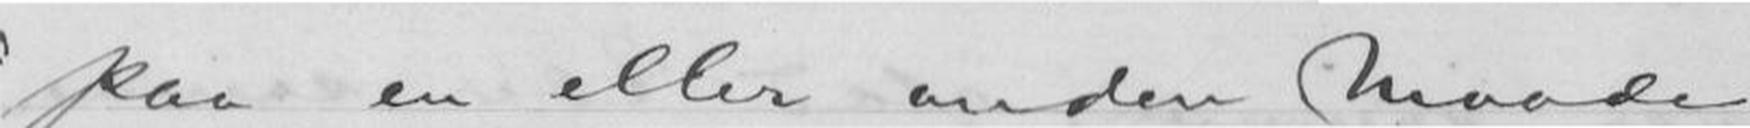paa en eller anden Maade
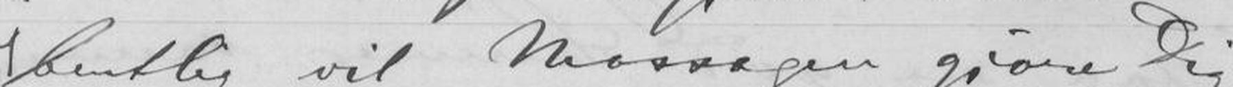bentlig vil Massagen gjøre Dig
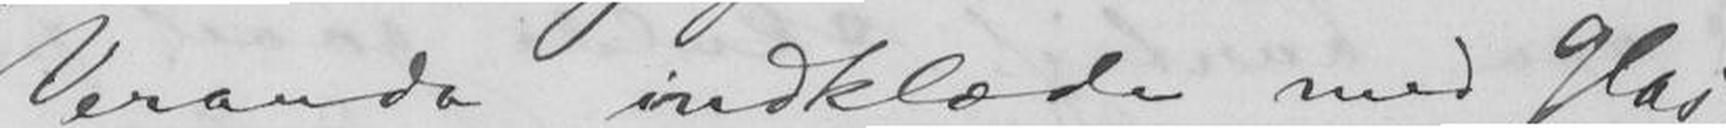Veranda indklæde med Glas,
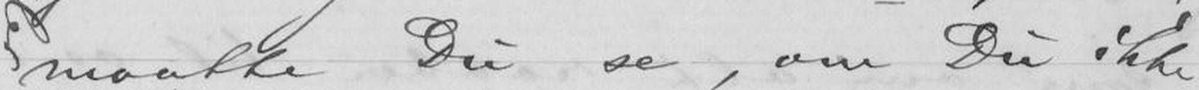maatte Du se, om Du ikke
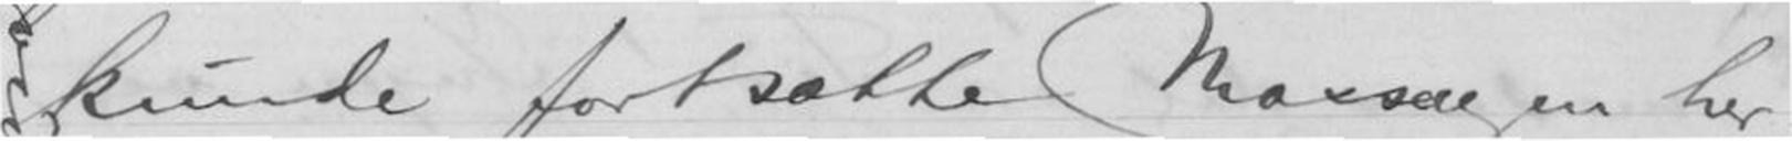kunde fortsætte Massagen her-
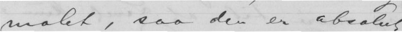malet, saa der er absolut
